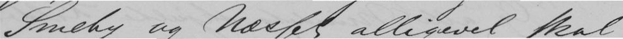Smeby og Næsset alligevel skal
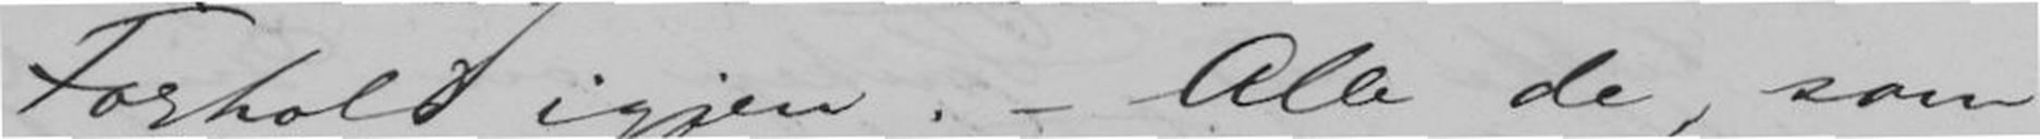Forhold igjen. - Alle de, som
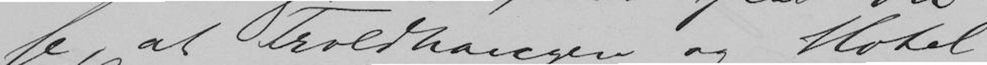se, at Troldhaugen og Hotel
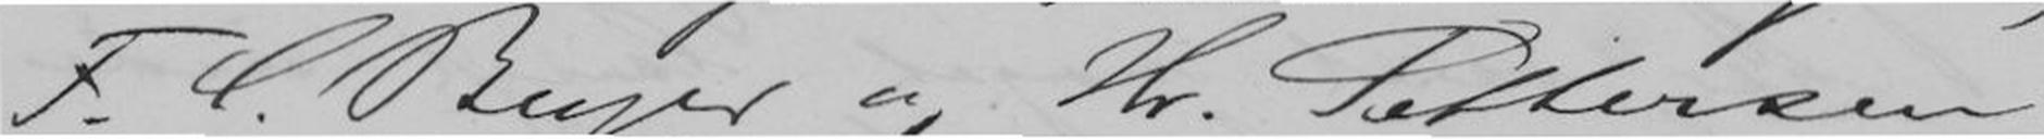F.C. Beyer og Hr. Pettersen,
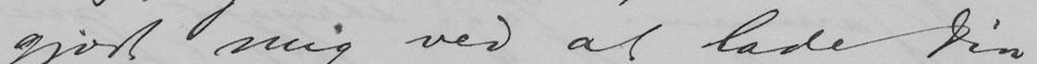gjort mig ved at lade Din
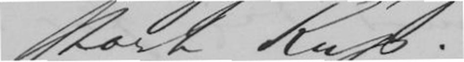stort Kup.
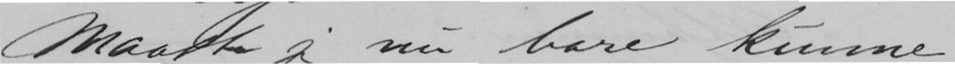Maaske Maatte? jeg nu bare kunne
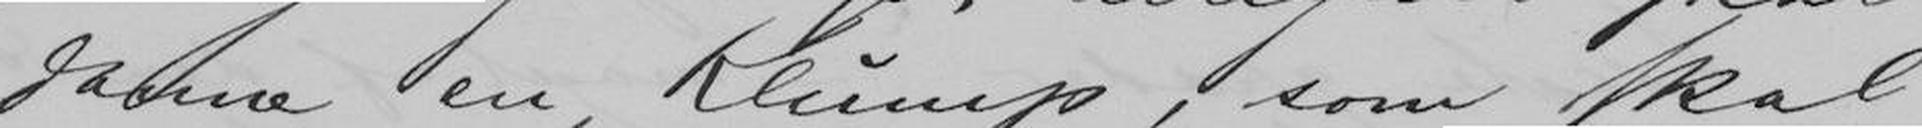danne en Klump, som skal
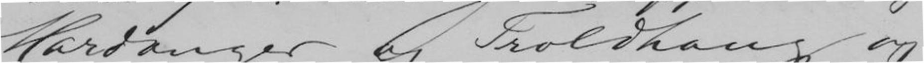og Hardanger og Troldhaug og
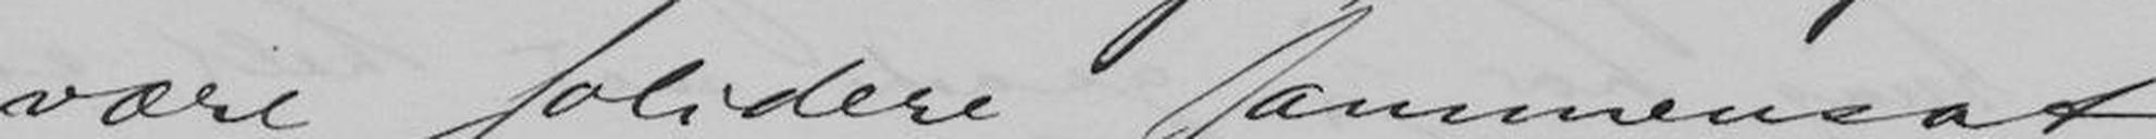være solidere sammensat
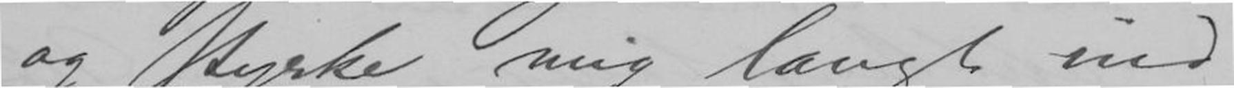og styrke mig langt ind
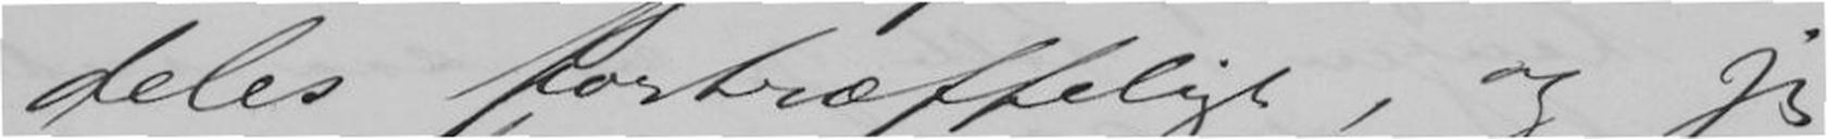deles fortræffeligt, og jeg
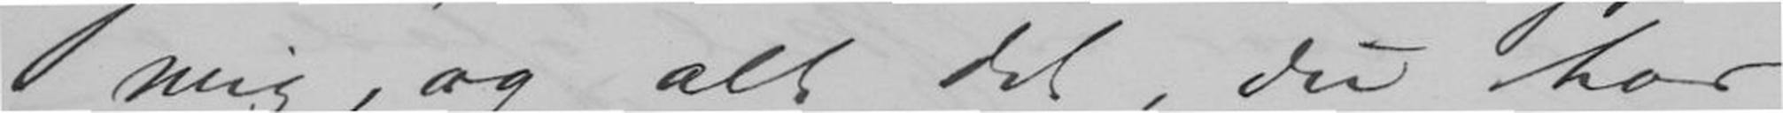mig, og alt det, Du har
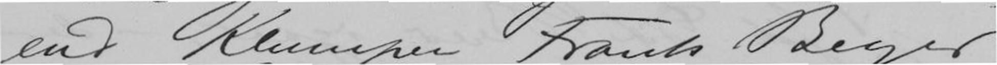end Klumpen Frants Beyer,
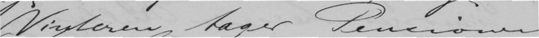Vinteren tager Pensionen
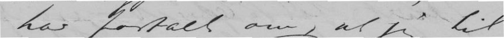jeg har fortalt om, at jeg til
